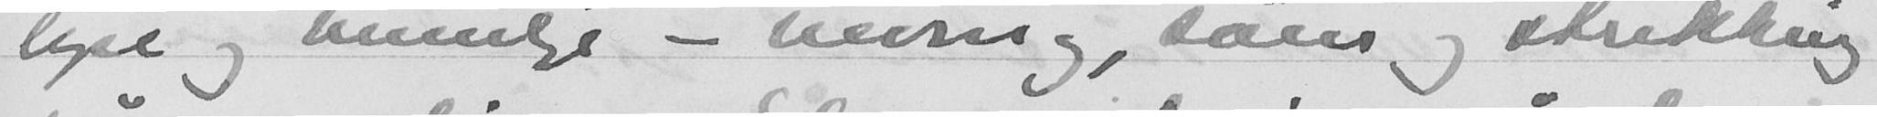lyse og kunnelige - neving, som og strikkeng
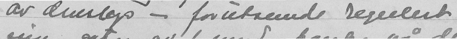av denslags - forutsemde regulert
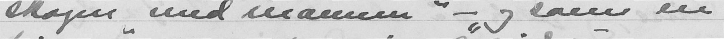skogen med mannen "- og sånn en
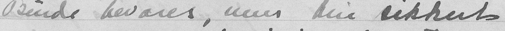Burde bevares, men blir sikkert
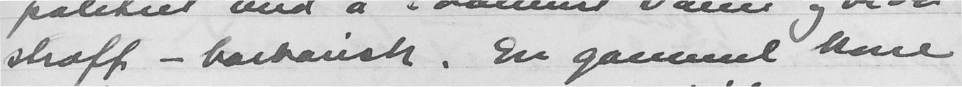straff - barbarisk. En gammel kone
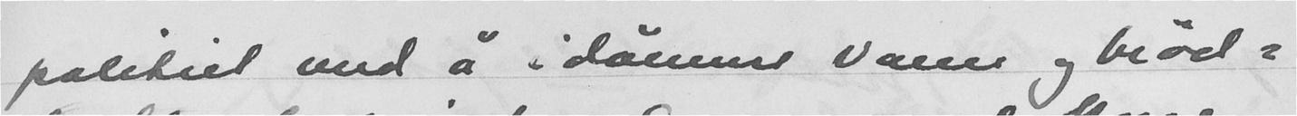politiet med å idømme vann og brød-
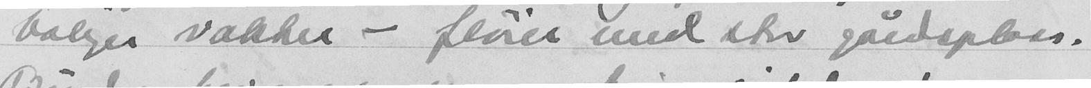balger vakker - fevser med stor gandsplass.
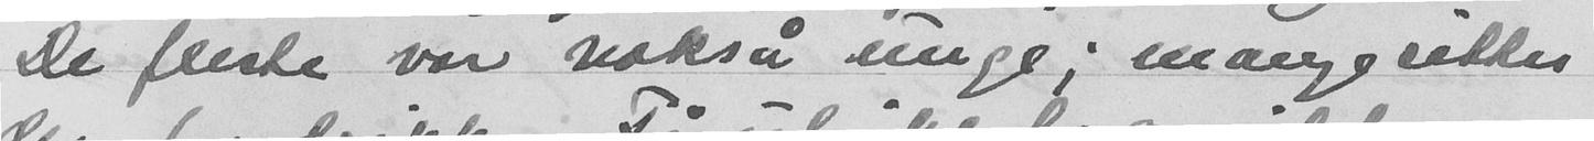De fleste var nokså unge; mange sitter
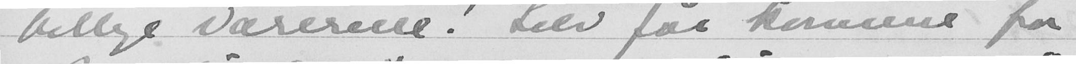billige varerene. Liev får kommune fra
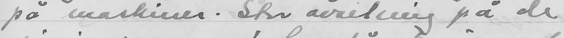på maskiner. Stor avsetning på de
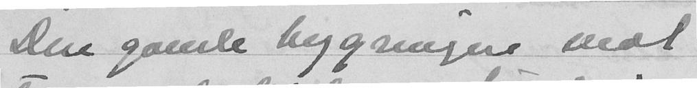Den gamle bygningen mot
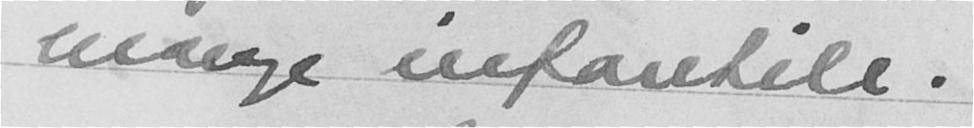mange infantile.
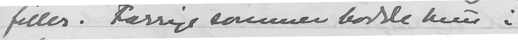filler. Forrige sommer bodde hun i
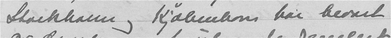Stockholm og Kjøbenhavn har bevart
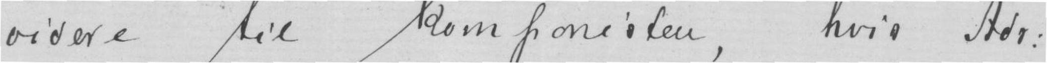videre til komponisten, hvis Adr.
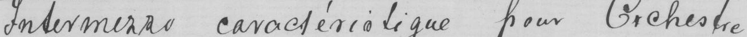Intermesso caradèriotiqne pour Grchestre
